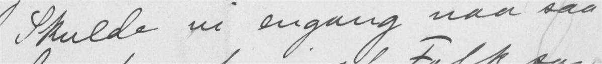Skulde vi engang naa saa
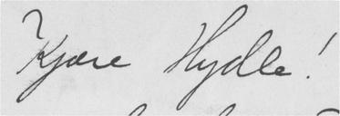Kjære Hydle!
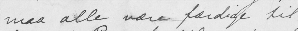maa alle være færdige til
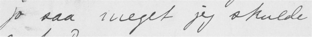jo saa meget jeg skulde
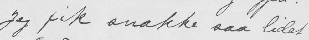Jeg fik snakke saa lidet
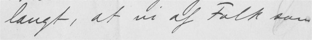langt, at vi af Folk som
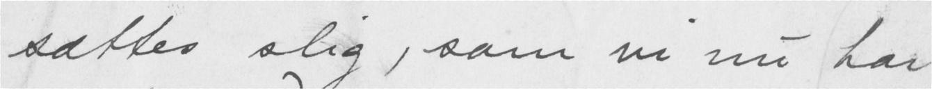sættes slig, som vi nu har
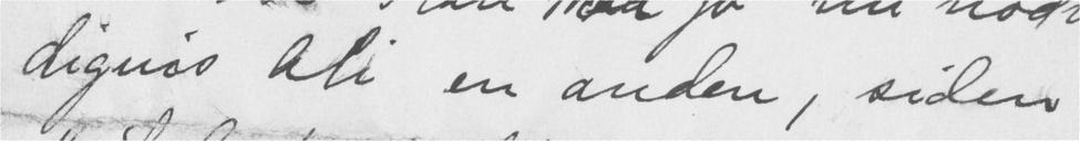digvis bli en anden, siden
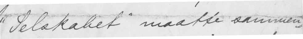"Selskabet" maatte sammen-
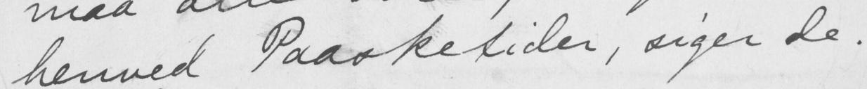henved Paasketider, siger de.
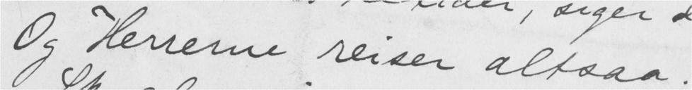Og Herrerne reiser altsaa.
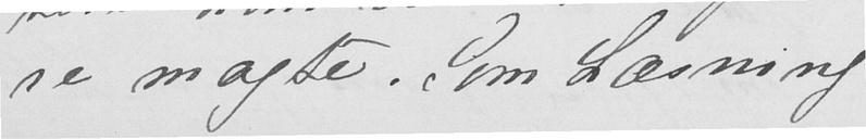re magte. Som Læsning
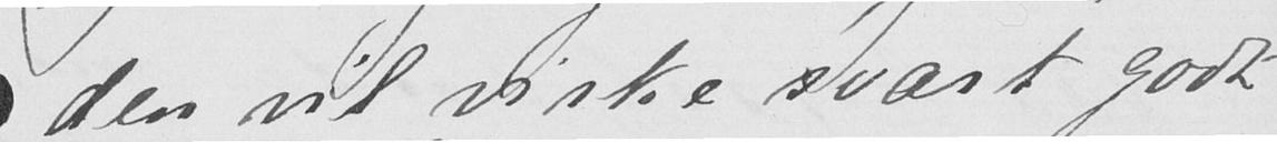den vil virke svært godt
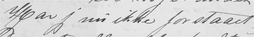Har jeg nu ikke forstaaet
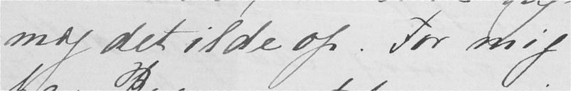mig det ilde op. For mig
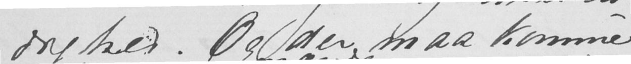dighed. Og der maa komme
